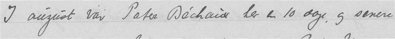I august var Pater Béchaux her en 10 dage, og senere
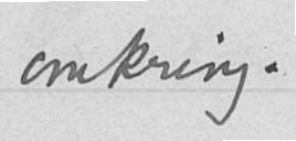omkring.
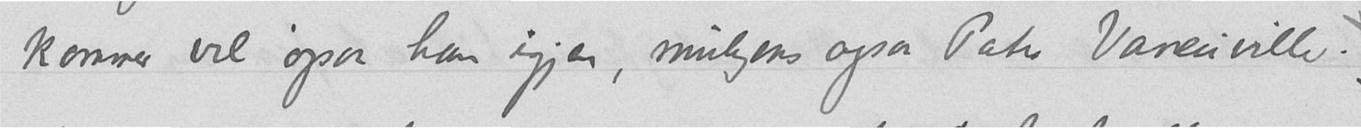kommer vel ogsaa han igjen, muligens ogsaa Pater Vaneuville.
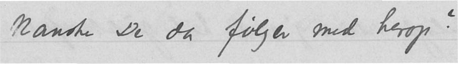kanske De da følger med herop?
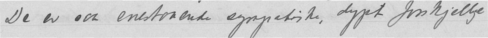De er saa enestaaende sympatiske, dypt forskjellige
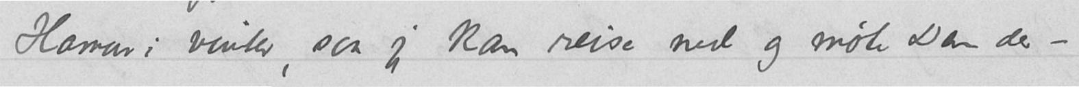Hamar i vinter, saa jeg kan reise ned og møte Dem der –
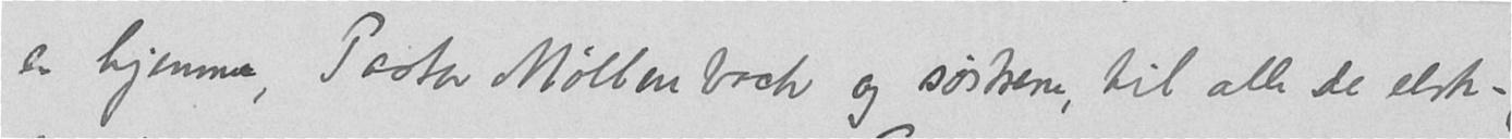er hjemme, Pastor Møllenbeck og søstrene, til alle de elsk-
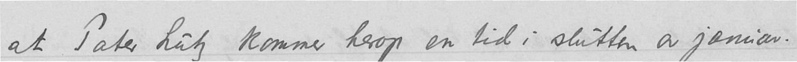at Pater Lutz kommer herop en tid i slutten av januar.
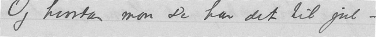Og hvordan mon De har det til jul –
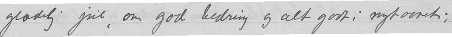glædelig jul, om god bedring og alt godt i nytaaret;
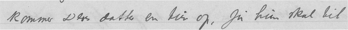kommer Deres datter en tur op, før hun skal til
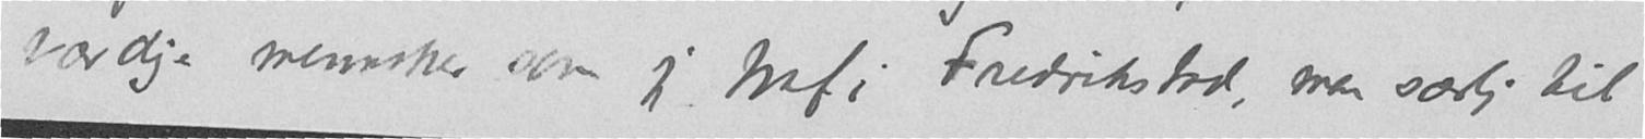værdige mennesker som jeg traf i Fredrikstad, men særlig til
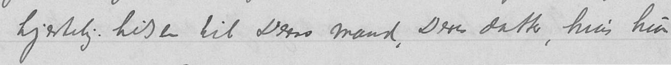hjertelig hilsen til Deres mand, Deres datter, hvis hun
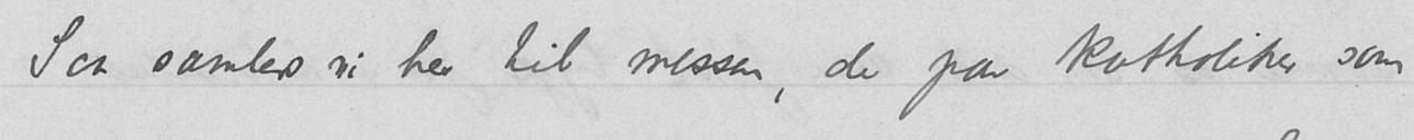Saa samles vi her til messen, de faa katholiker som
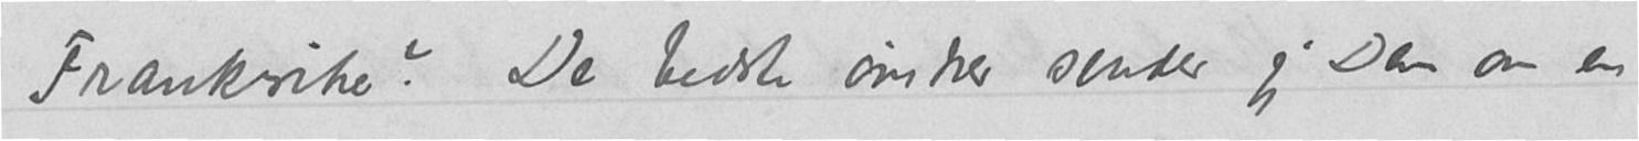Frankrike? De bedste ønsker sender jeg Dem om en
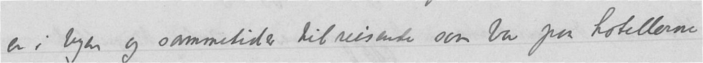er i byen og sommetider tilreisende som bor paa hotellerne
